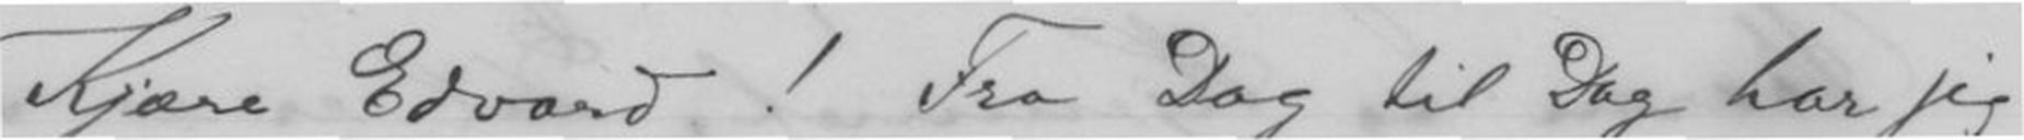Kjære Edvard!Fra Dag til Dag har jeg
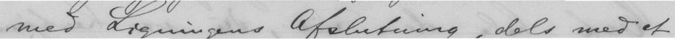med Ligningens Afslutning, dels med et
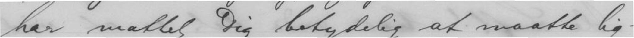har mattet Dig betydelig at maatte lig-
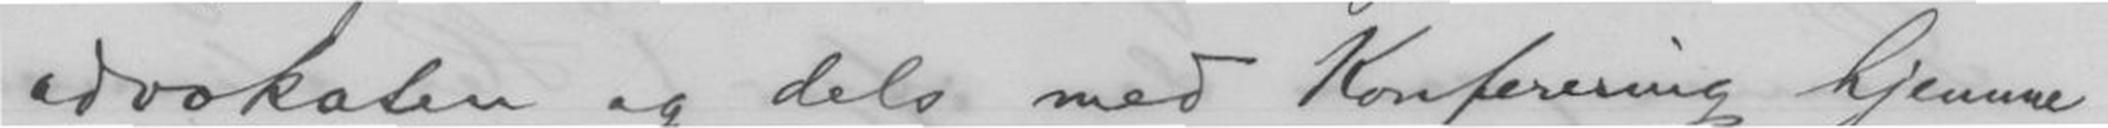advokaten og dels med Konferering hjemme
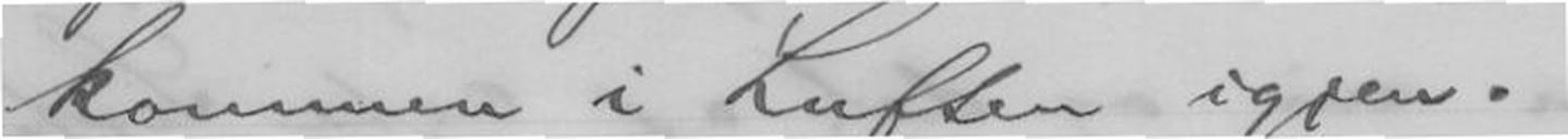kommen i Luften igjen.
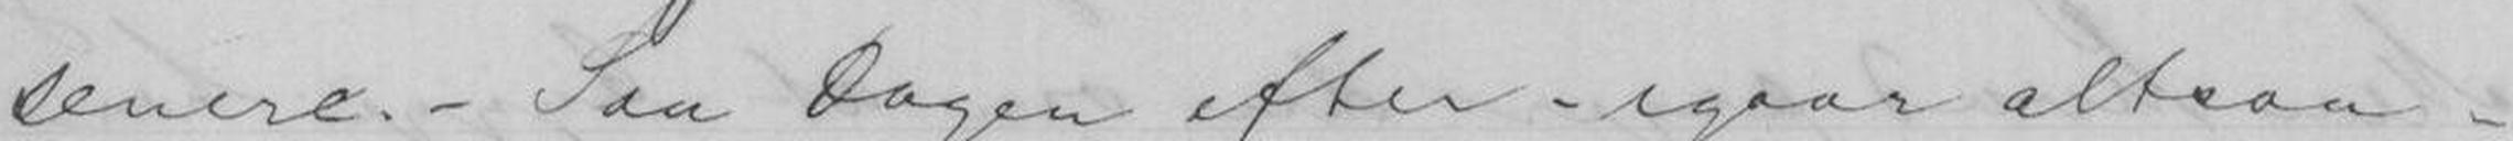senere. - Saa Dagen efter - igaar altsaa -
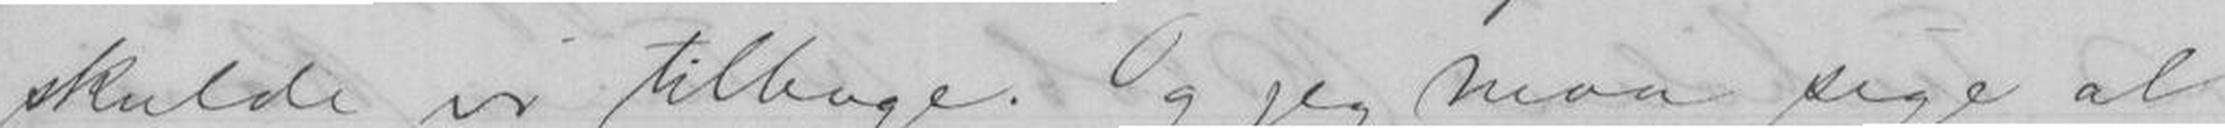skulde vi tilbage. Og jeg maa sige at
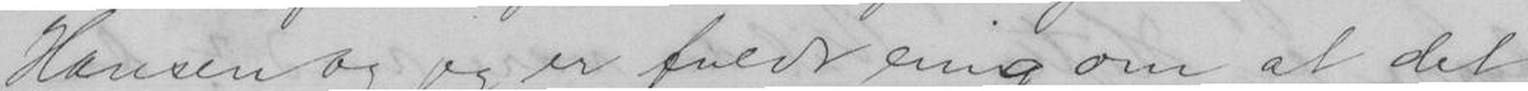Hansen og jeg er fuldt enig om at det
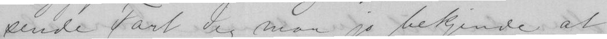sende Fart Jeg maa jo bekjende at
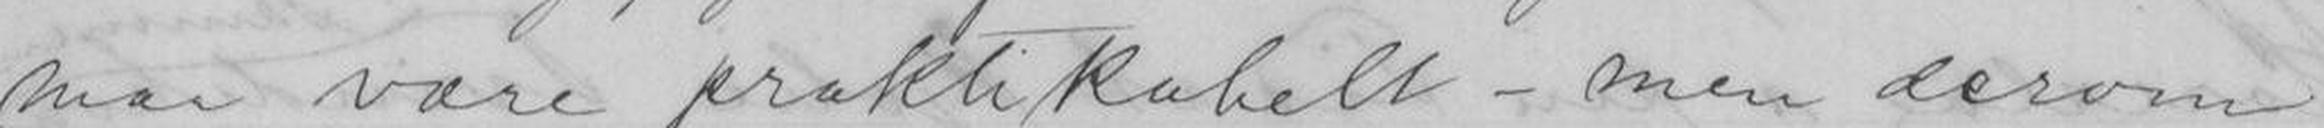maa være praktikabelt - men derom
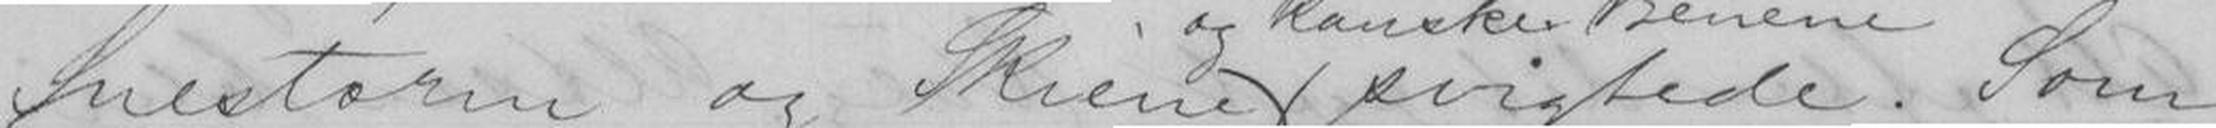Snestorm og Skiene svigtede. Som
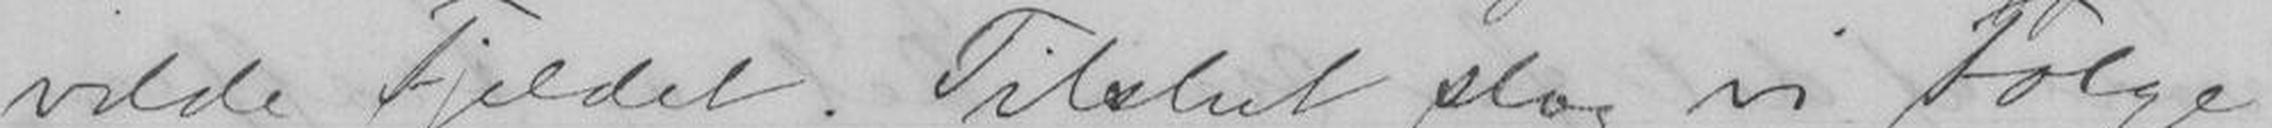vilde Fjeldet. Tilslut slog vi Følge
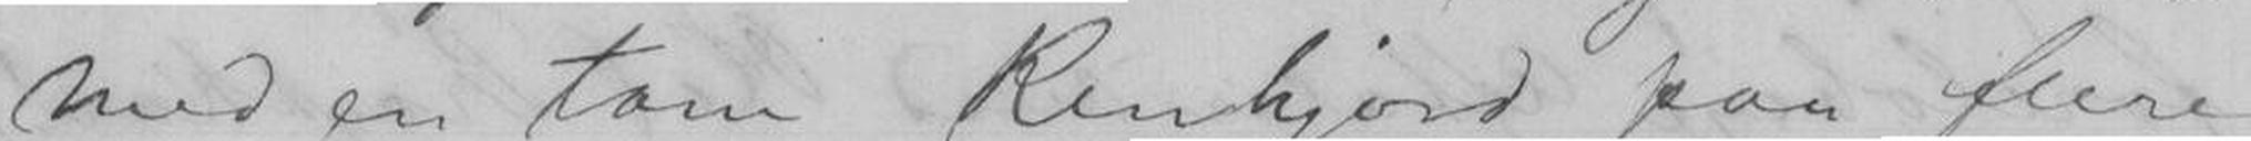med en tam Renhjord paa flere
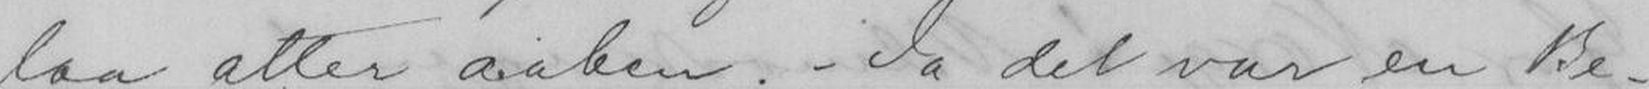laa atter aaben. - Ja det var en Be-
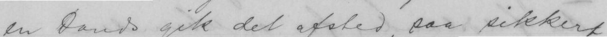en Dands gik det afsted, saa sikkert
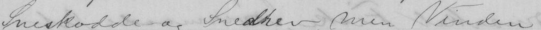Sneskodde og Snedrev, men Vinden
